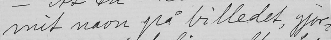mit navn på billedet, gjor-
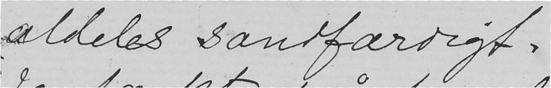aldeles sandfærdigt.
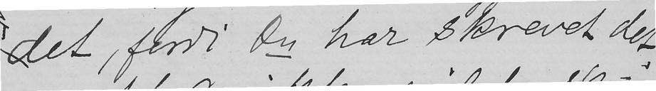det, fordi Du har skrevet det
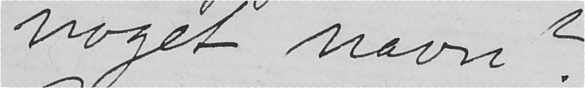noget navn?
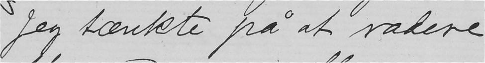Jeg tænkte på at radere
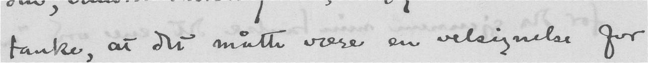tanke, at det måtte være en velsignelse for
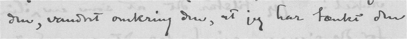den, vandret omkring den, at jeg har tænkt den
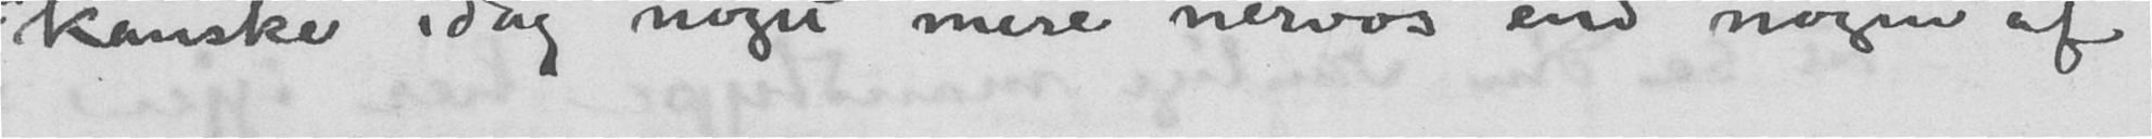kanske idag noget mere nervøs end nogen af
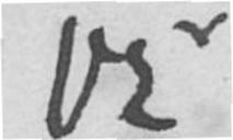jeg
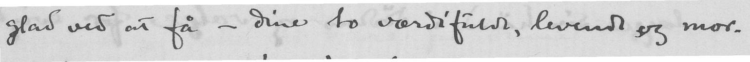glad ved at få - dine to værdifulde, levende og mor-
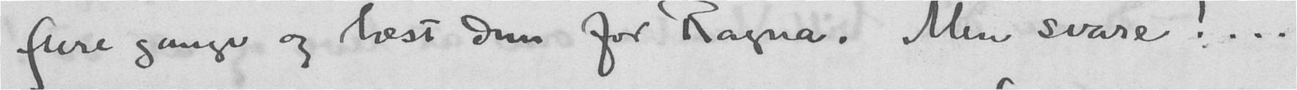flere gange og læst dem for Ragna. Men svare!...
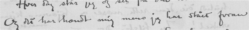Og det har hændt mig mens jeg har stået foran
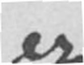er
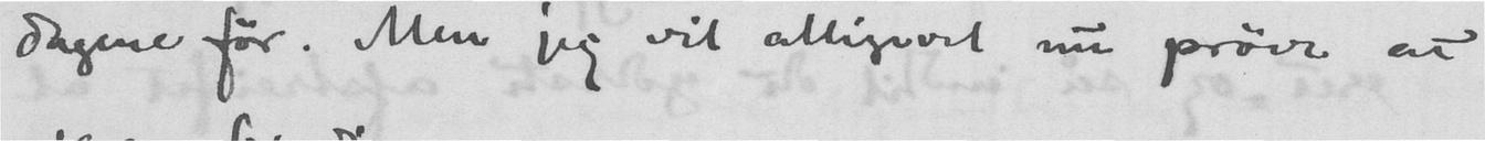dagene før. Men jeg vil alligevel nu prøve at
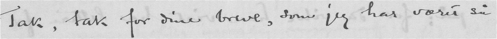Tak, tak for dine breve, som jeg har været så
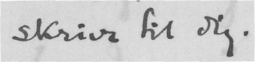skrive til dig.
